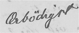Ærbødigst
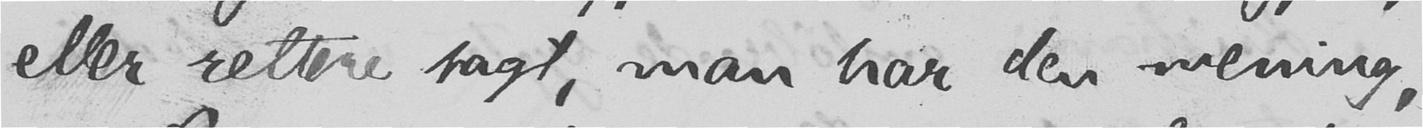eller rettere sagt, man har den mening,
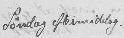Søndag eftermiddag.
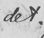det.
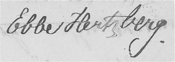Ebbe Hertzberg.
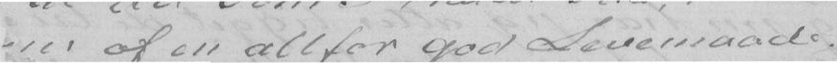af en allfor god Levemaade
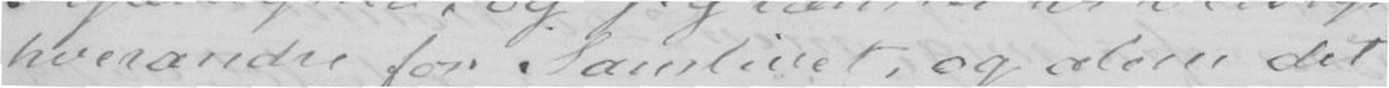hverandre for Samlivet, og alene det
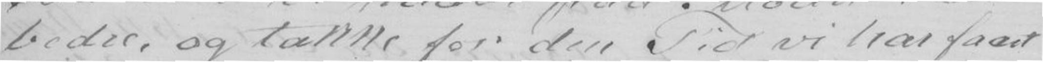bedre, og takke for den Tid vi har faaet
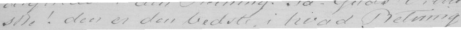ske! den er den bedste i hvad Retning
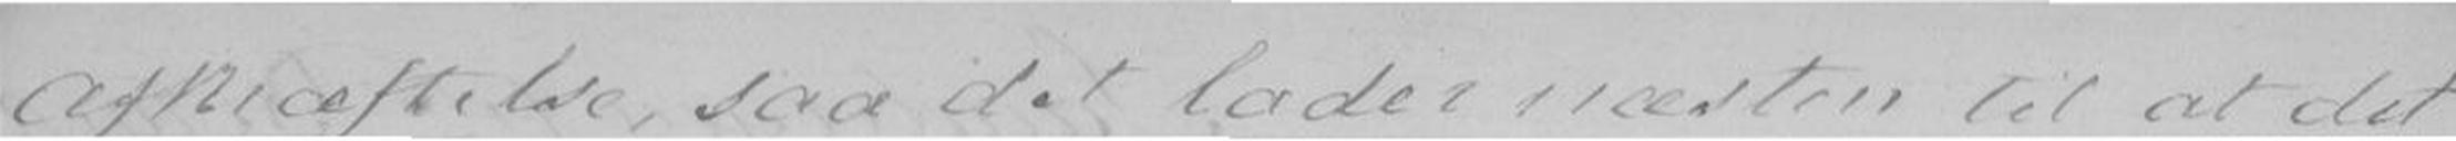Afkræftelse, saa det lader næsten til at det
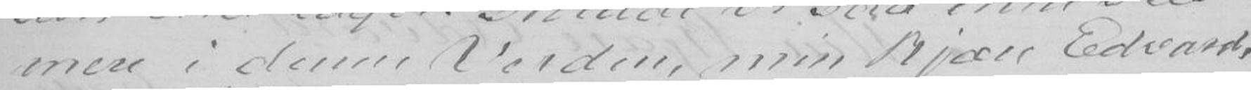mere i denne Verden, min kjære Edvard,
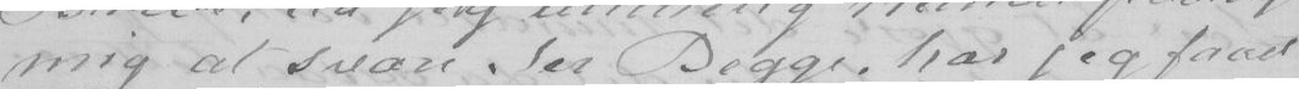mig at svare Jer Begge, har jeg faaet
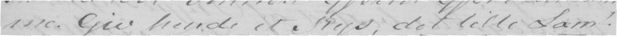me. Giv hende et Kys, det lille Lam
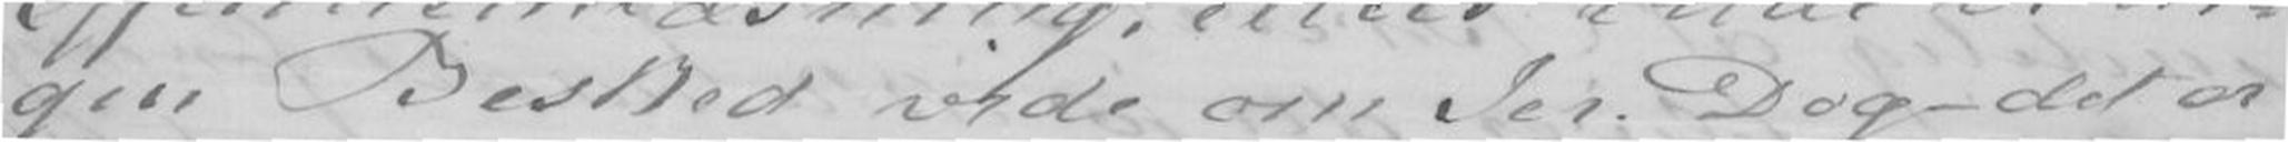gen Besked vide om Jer. Dog - det er
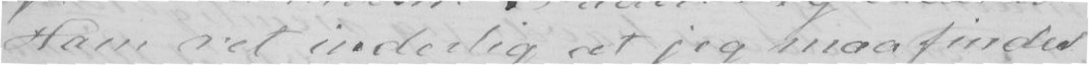Ham ret inderlig at jeg maa findes
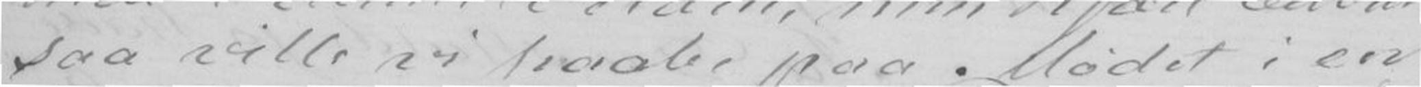saa ville vi haabe paa Mødet i en
